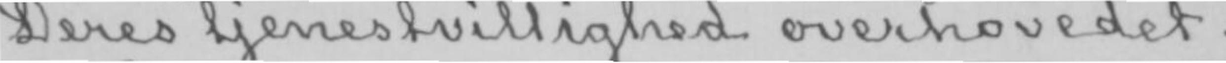Deres tjenestevillighed overhovedet.
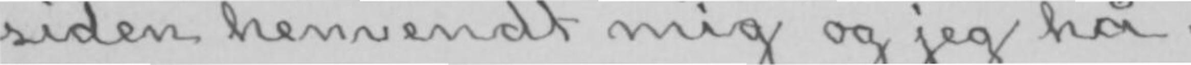siden henvendt mig og jeg hå-
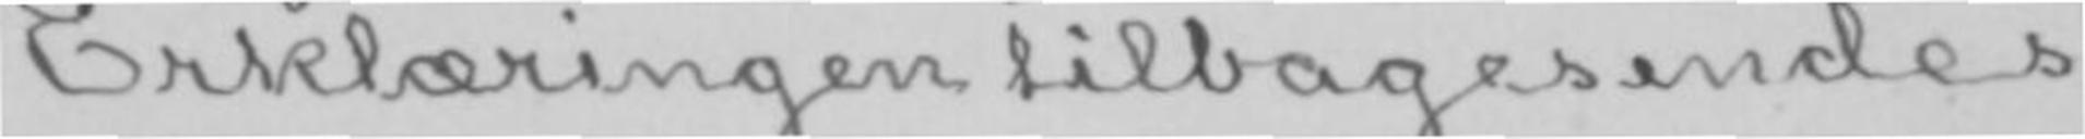Erklæringen tilbagesendes
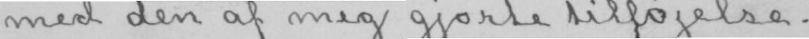med den af mig gjorte tilføjelse.
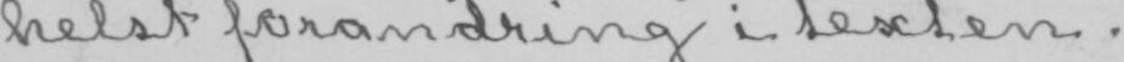helst forandring i texten.
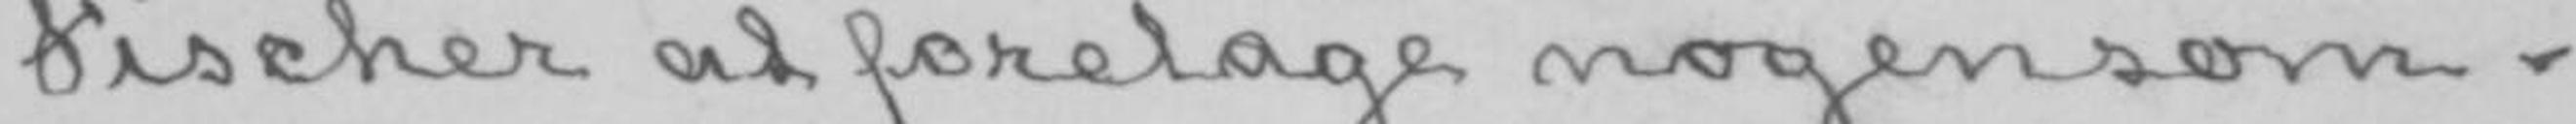Fischer at foretage nogensom-
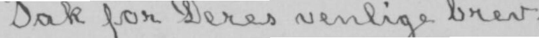Tak for Deres venlige brev,
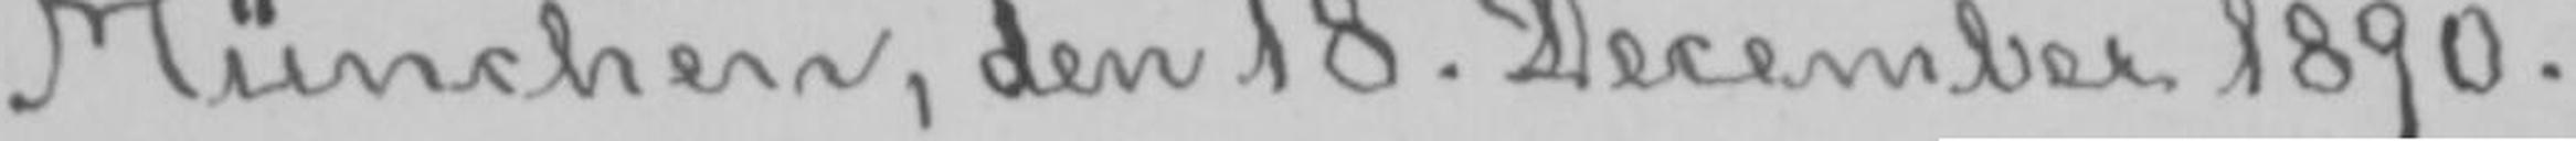München, den 18. December 1890.
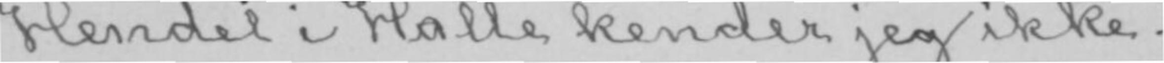Hendel i Halle kender jeg ikke.
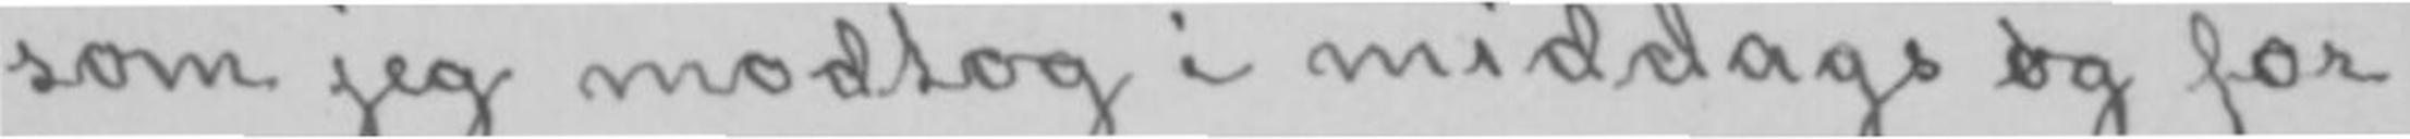som jeg modtog i middags og for
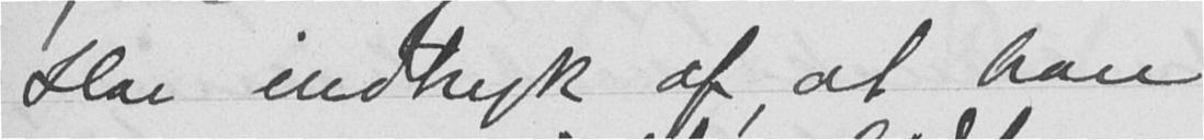Har indtryk af at han
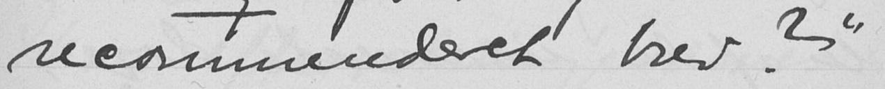recommenderet brev?"
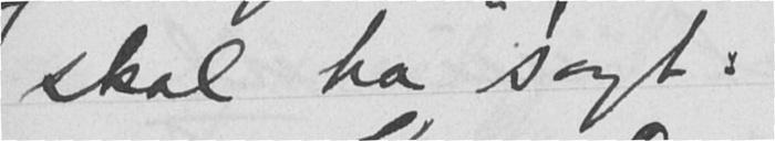skal ha sagt:
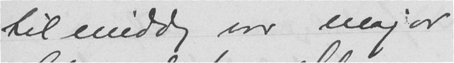til middag var major
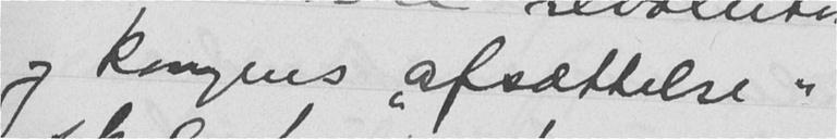og kongens "afsættelse"
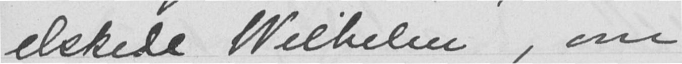elskede Wilhelm, om
